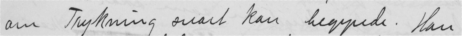om Trykning snart kan begynde. Han
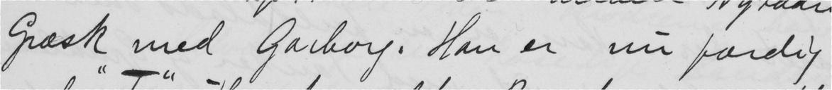Græsk med Garborg. Han er nu færdig
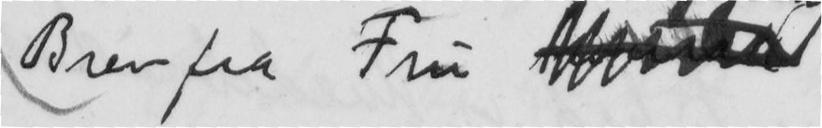Brev fra Fru
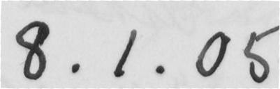8.1.05
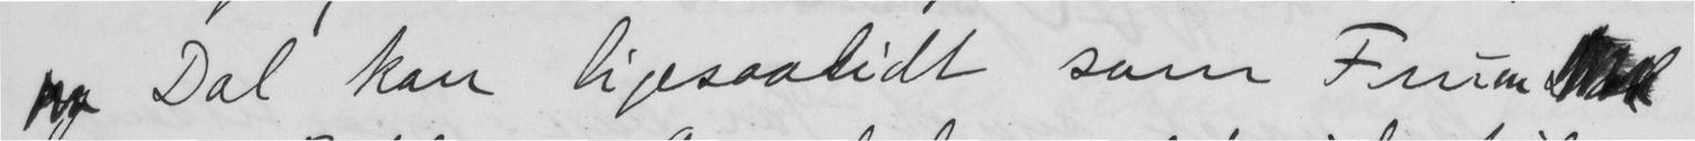Dal kan ligesaalidt som Fruen
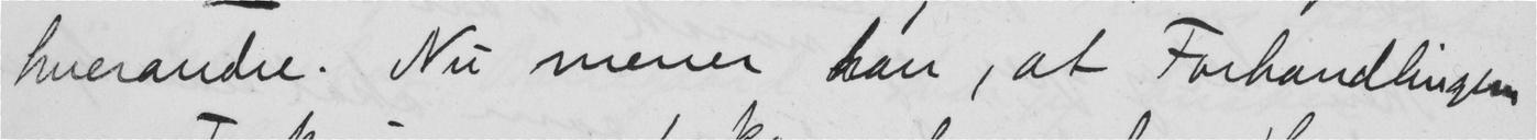hverandre. Nu mener han, at Forhaudlingen
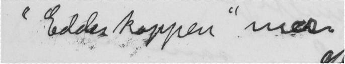"Edderkoppen" mer.
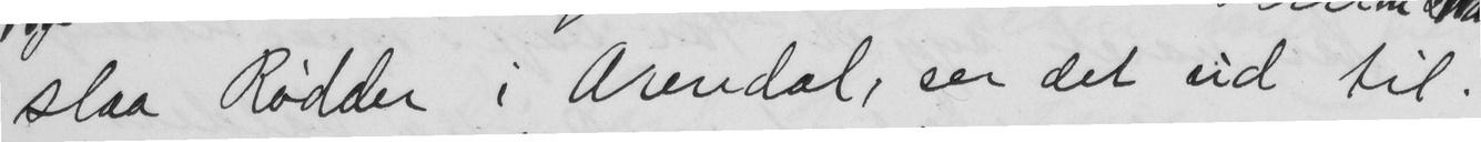slaa Rødder i Arendal, ser det ud til.
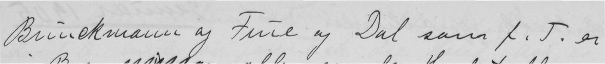Brinckmann og Frue og Dal som f. T. er
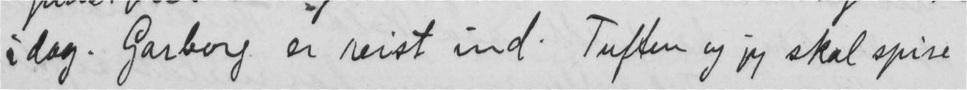idag. Garborg er reist ind. Tuften og jeg skal spise
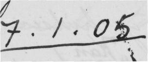7.1.05
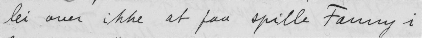lei over ikke at faa spille Fanny i
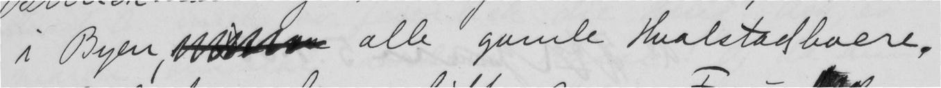i Byen,  alle gamle Hvalstadboere.
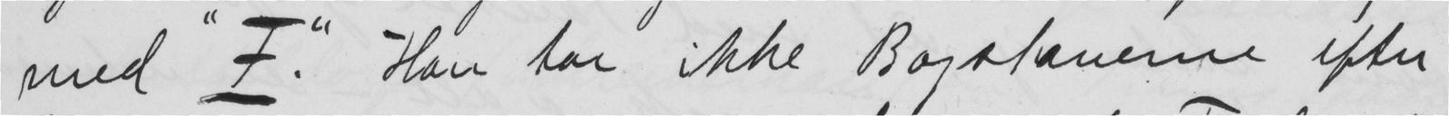med "E". Han tar ikke Bogstaverne efter
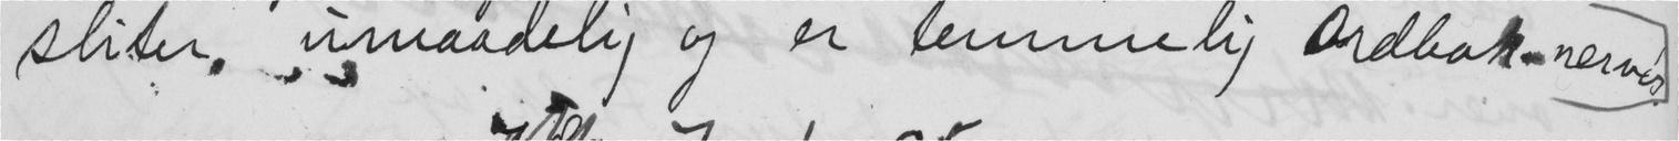sliter, umaadelig og er temmelig Ordbok-nervøs
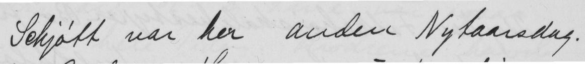Schjøtt var her anden Nytaarsdag.
